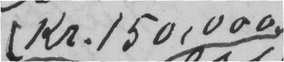Kr. 150,000.
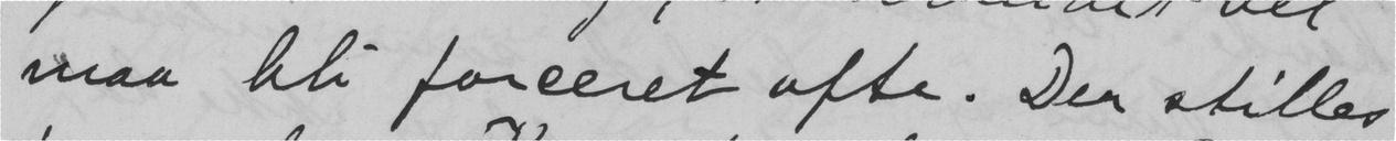maa bli forceret ofte. Der stilles
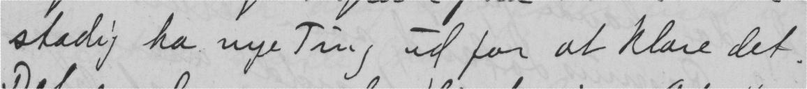stadig ha nye Ting ud for at klare det.
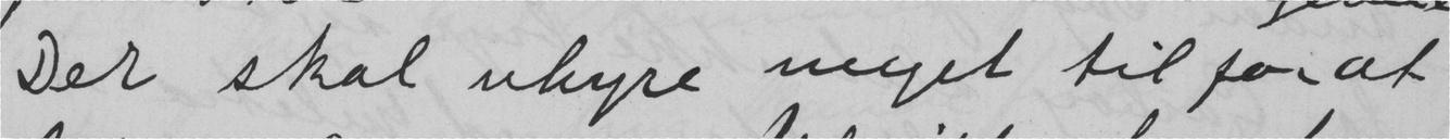Der skal uhyre meget til for at
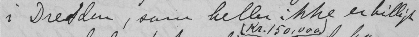i Dresden, som heller ikke er billigt
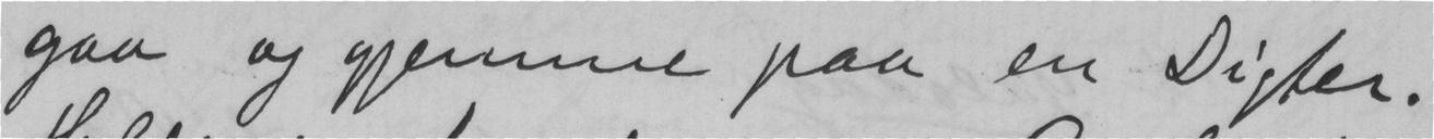gaa og gjemme paa en Digter.
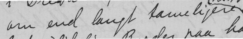om end langt tarveligere
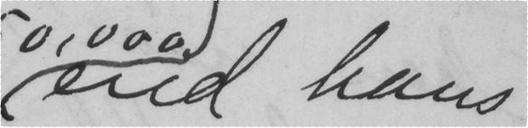end hans
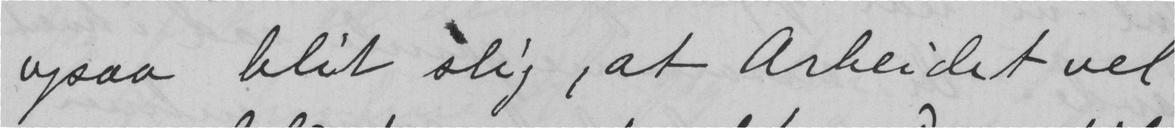ogsaa blit slig, at Arbeidet vel
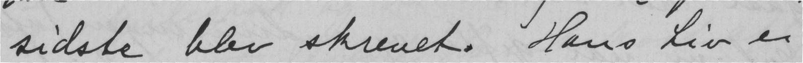sidste blev skrevet. Hans Liv er
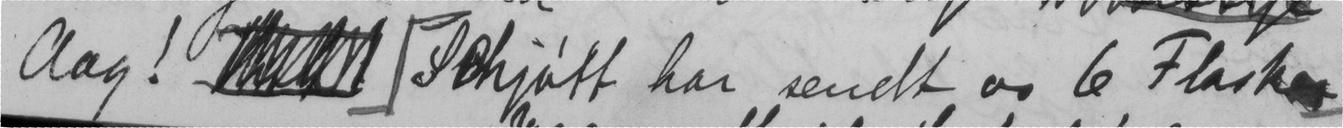Aag!  Schjøtt har sendt os 6 Flasker
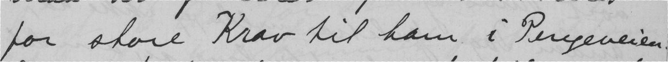for store Krav til ham i Pengeveien.
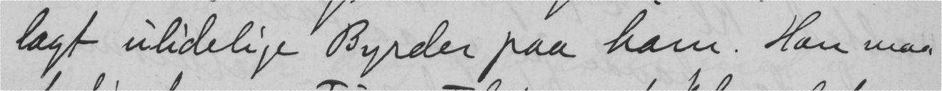lagt ulidelige Byrder paa ham. Han maa
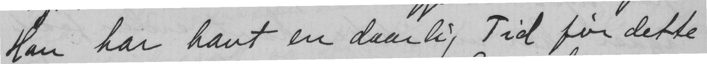Han har havt en daarlig Tid før dette
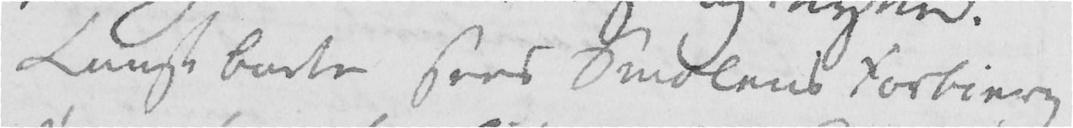Langt borte sees Smølens Forbierg
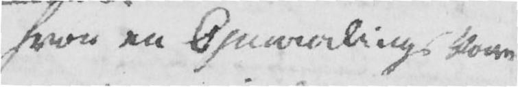hvor en Opmaalings Vare
